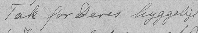Tak for Deres hyggelige
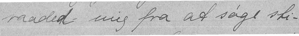raaded mig fra at søge sti-
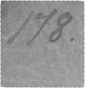178.
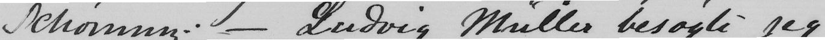Schønning. -- Ludvig Müller besøgte jeg
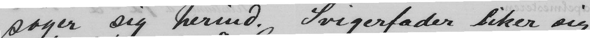søger sig herind. Svigerfader liker sig
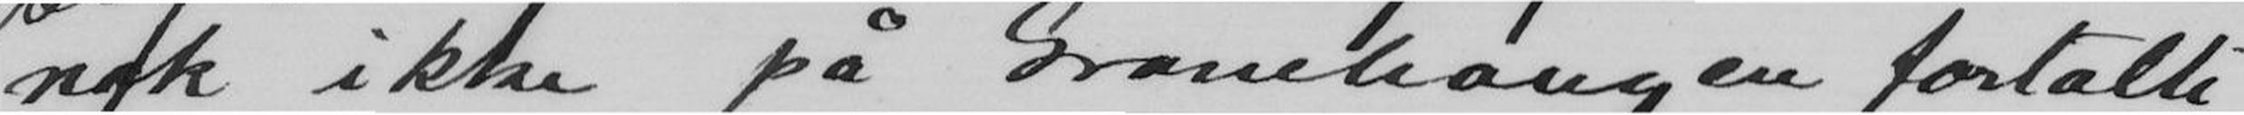nok ikke på Granehaugen fortalte
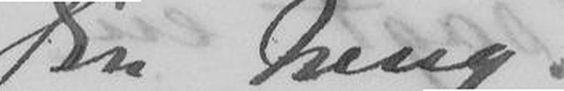Din heng.
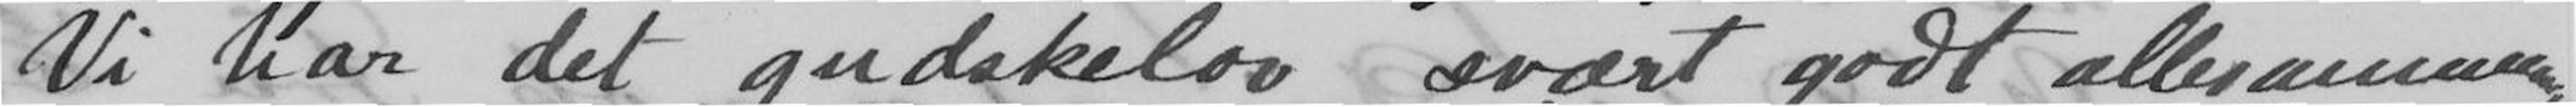Vi har det gudskelov svært godt allesammen.
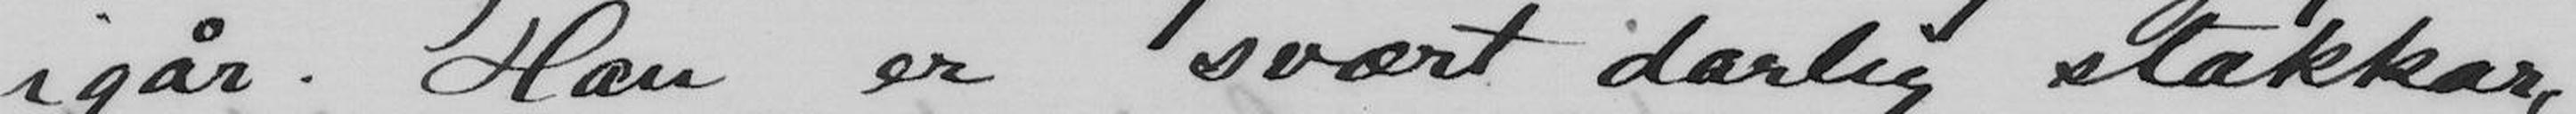igår. Han er svært dårlig stakkar,
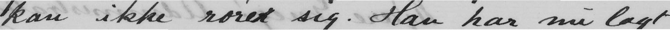kan ikke røre sig. Han har nu lagt
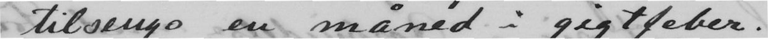tilsengs en måned i gigtfeber.
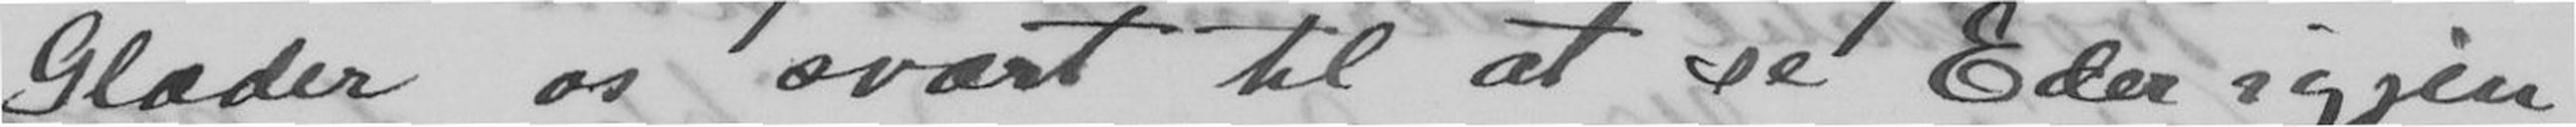Glæder os svært til at se Eder igjen.
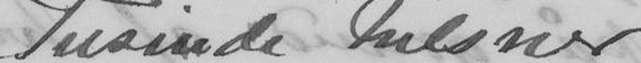Tusinde hilsner
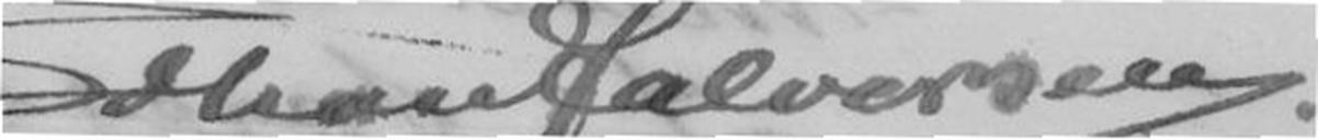Johan Halvorsen.
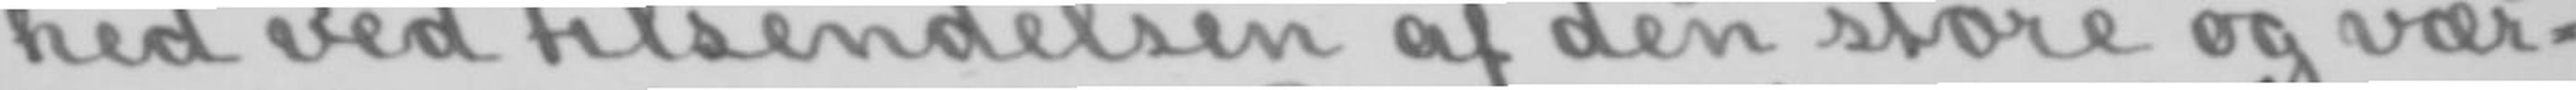hed ved tilsendelsen af den store og vær-
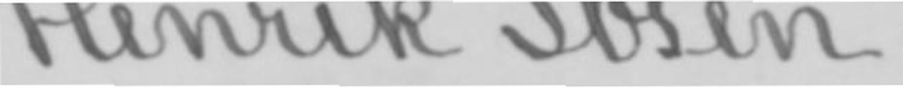Henrik Ibsen.
